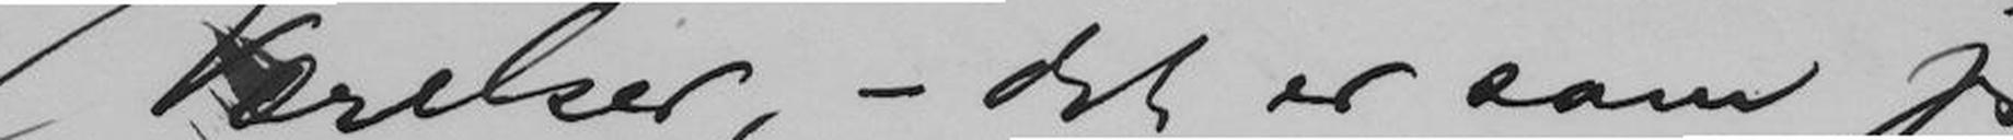Værelser, - det er som jeg
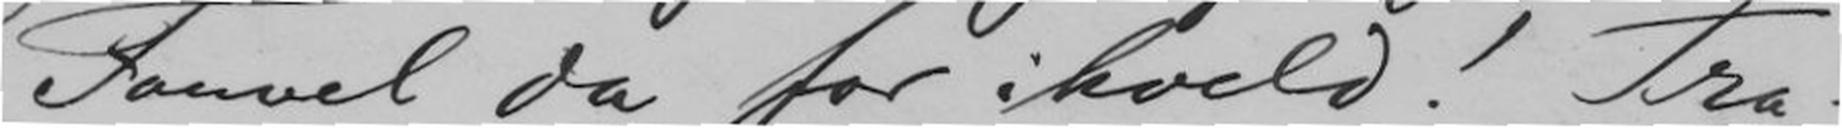Farvel da for ikveld! Tra -
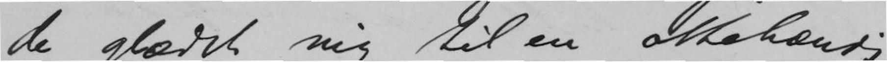de glædet mig til en ottehændig
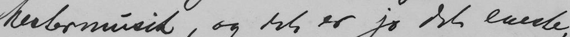kestermusik, og det er jo det eneste,
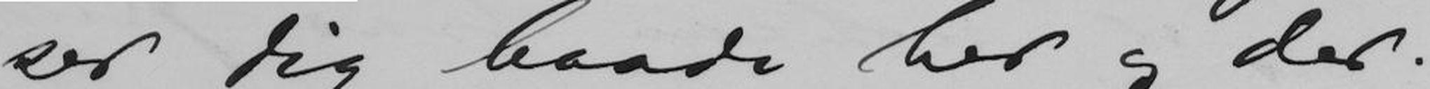ser dig baade her og der.
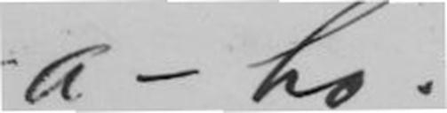- a - ho!
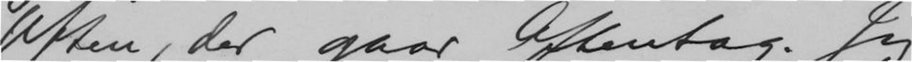Aften, der gaar Aftentog. Jeg
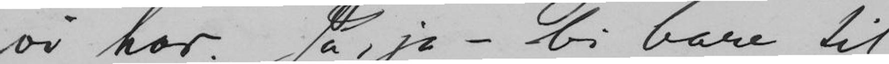vi har. Ja, ja - bi bare til
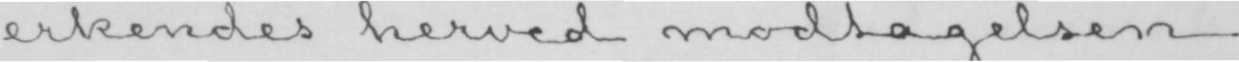erkendes herved modtagelsen
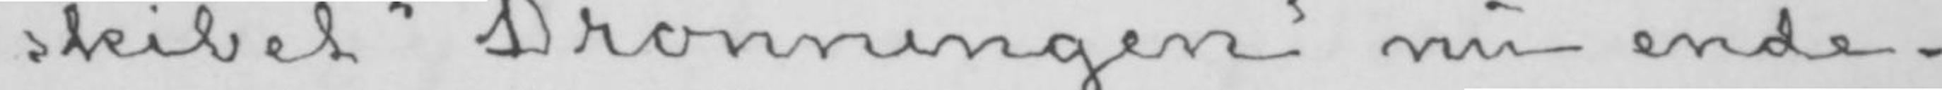skibet «Dronningen» nu ende-
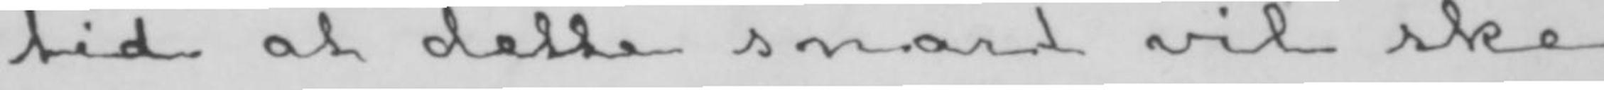lertid at dette snart vil ske
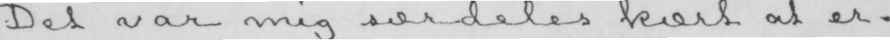Det var mig særdeles kært at er-
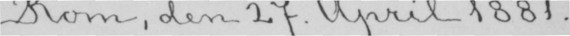Rom, den 27. April 1881.
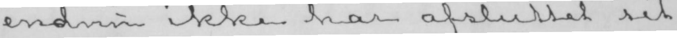endnu ikke har afsluttet sit
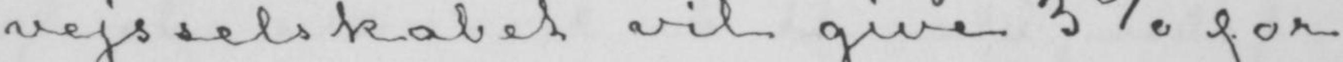vejsselskabet vil give 3% for
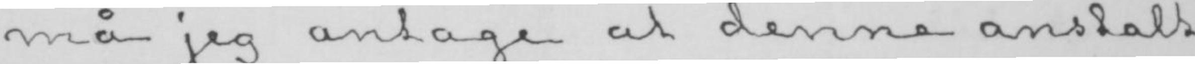må jeg antage at denne anstalt
